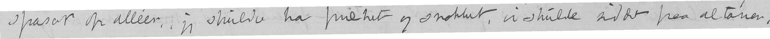spasert op alléen,, jeg skulde ha præket og snakket, vi skulde siddet paa altanen,
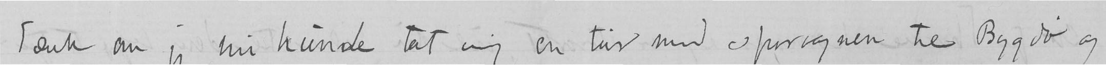Tænk om jeg nu kunde tat mig en tur med sporvognen til Bygdø og
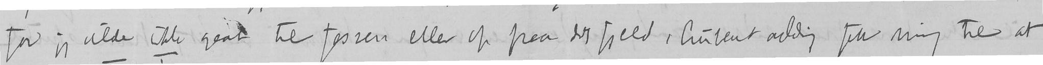for jeg vilde ikke gaat til fossen eller op paa det fjeld, Aubert aldrig fik mig til at
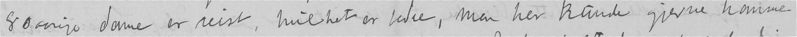80 aarige dame er reist, hvilket er bedre, men her kunde gjerne komme
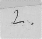2.
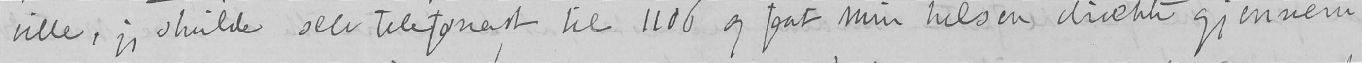ville, jeg skulde selv telefoneret til 1106 og faat min hilsen direkte gjennem
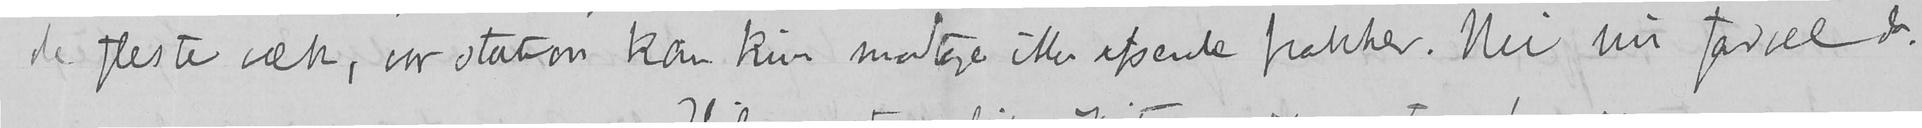de fleste væk, vor station kan kun modtage ikke afsende pakker. Nei nu farvel da.
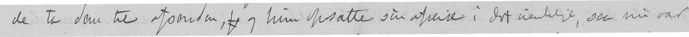de ta dem til afsender, og og hun opsatte sin afreise i det uendelige, saa nu var
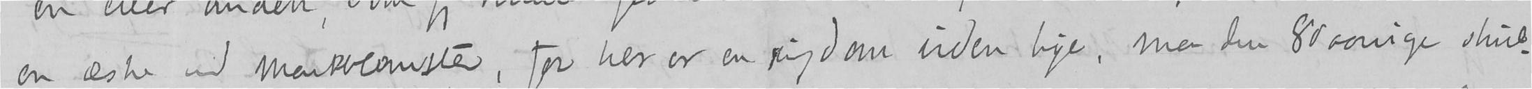en æske med markblomster, for her er en rigdom uden lige, men den 80 aarige skul-
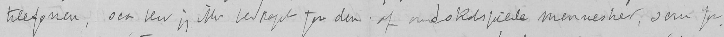telefonen, saa blev jeg ikke bedraget for den af ondskabsfulde mennesker, som for-
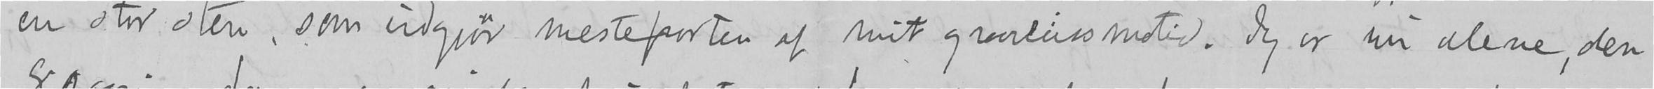en stor sten, som udgjør mesteparten af mit graaveirsmotiv. Jeg er nu alene, den
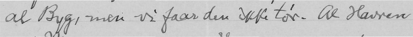al Byg, men vi faar den ikke tør. Al Havren
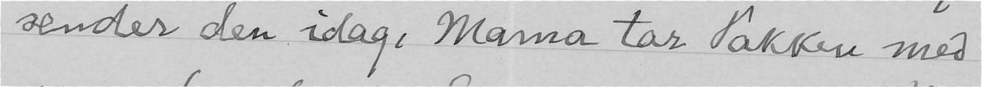sender den idag, Mama tar Pakken med
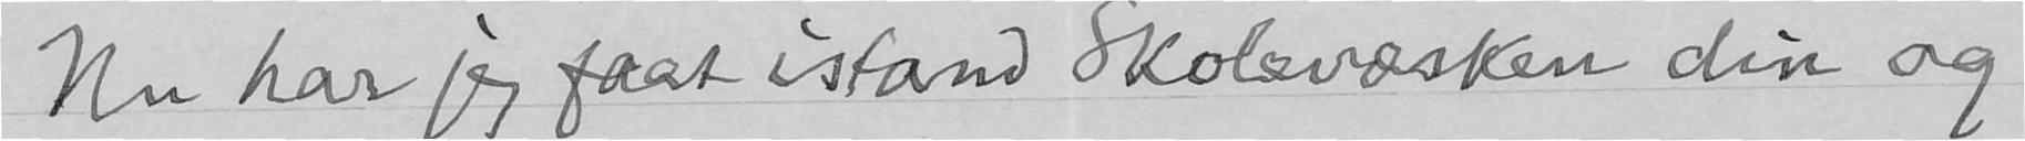Nu har jeg faat istand Skolevæsken din og
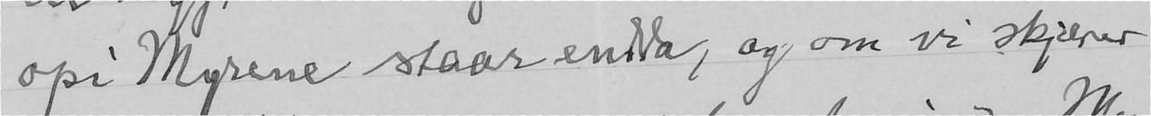opi Myrene staar endda, og om vi skjærer
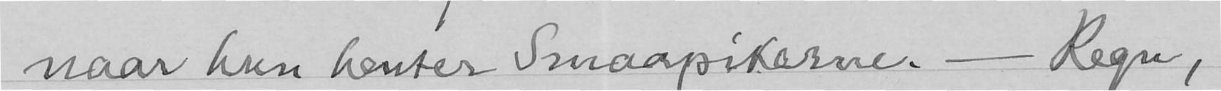naar hun henter Smaapikerne. - Regn,
